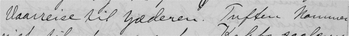Vaarreise til Jæderen. Tuften kommer
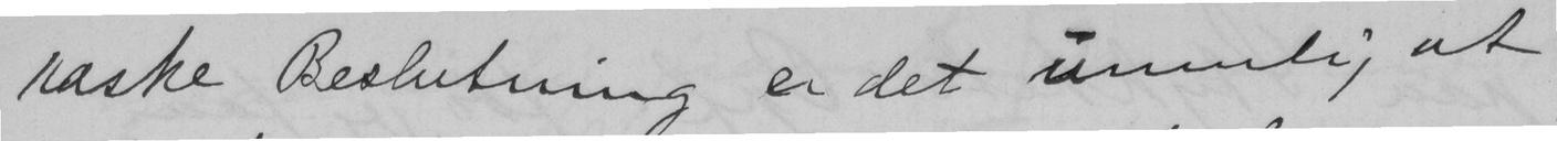raske Beslutning er det umulig at
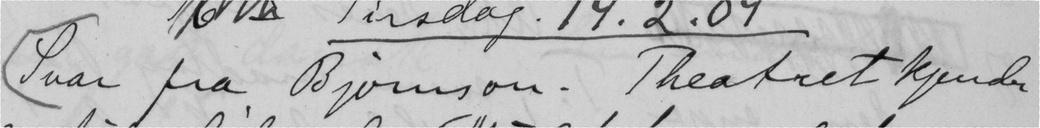Svar fra Bjørnson. Theatret kjender
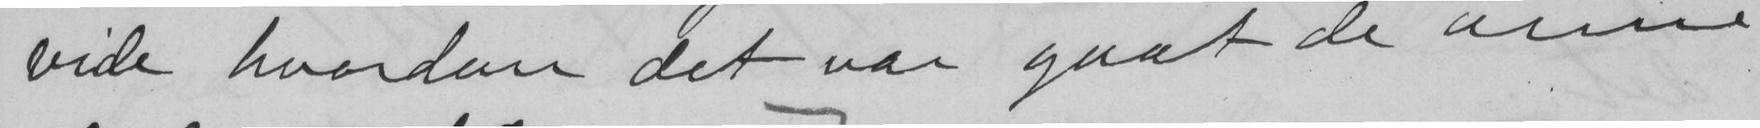vide hvordan det var gaat de arme
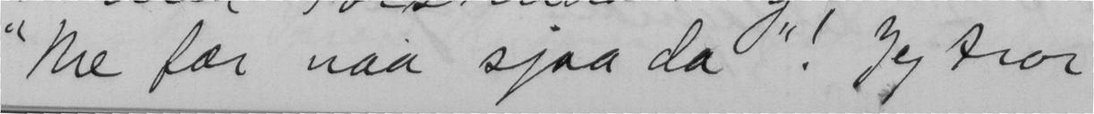"Me fær naa sjaa da"! Jeg tror
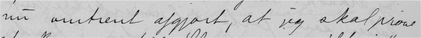nu omtrent afgjort, at jeg skal prøve
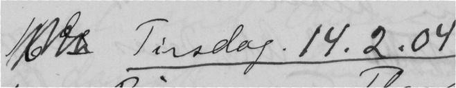Tirsdag. 14.2.04
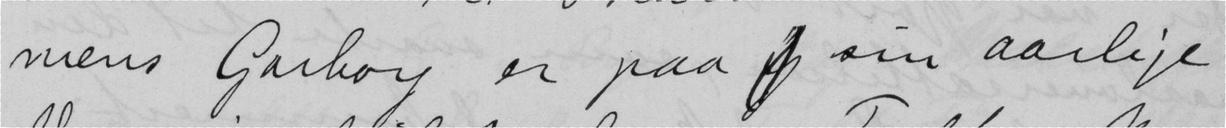mens Garborg er paa  sin aarlige
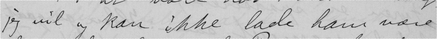jeg vil og kan ikke lade ham være
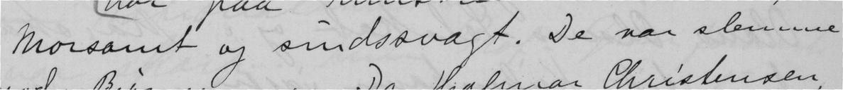Morsomt og sindssvagt. De var slemme
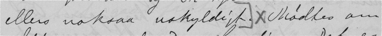ellers noksaa uskyldigt. x Mødtes om
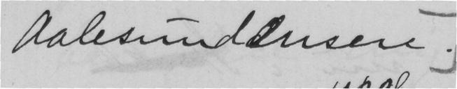Aalesundænsere.
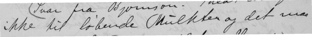ikke til løbende Mulkter og det maa
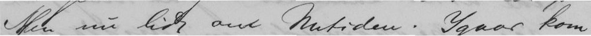- Men nu lidt om Nutiden. Igaar kom
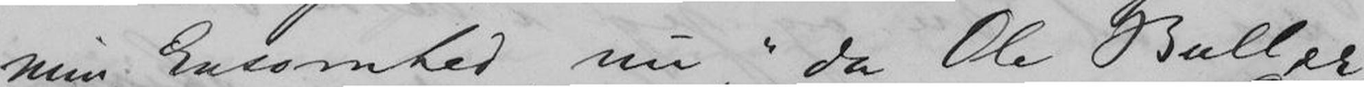min Ensomhed nu, "da Ole Bull er
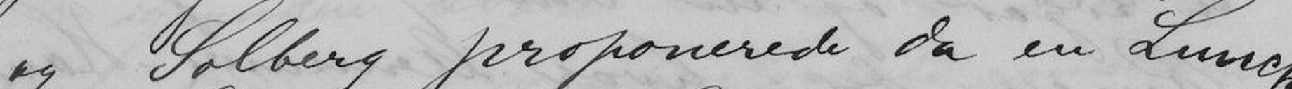og Solberg Proponerede da en Lunch
